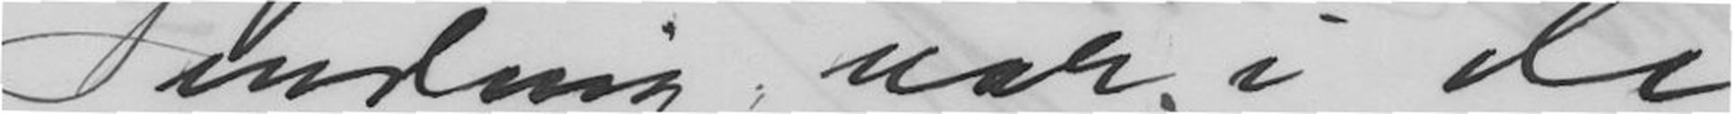Sinding var i de
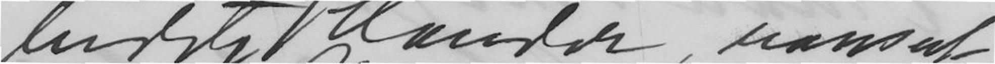bedste Hænder, uanseet
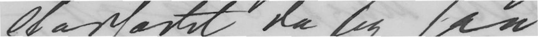storfartet da jeg saa
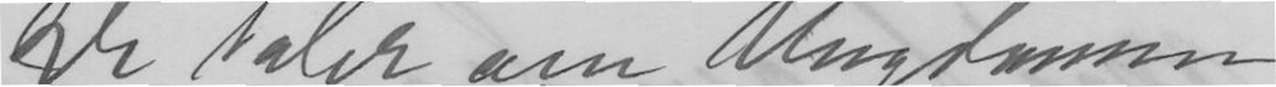De taler om Ungdommen
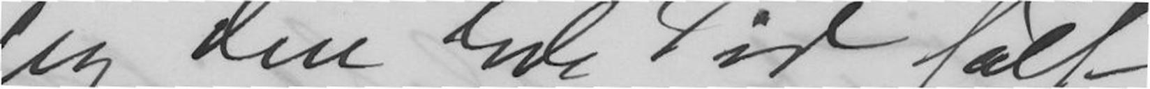jeg den hele Tid følt
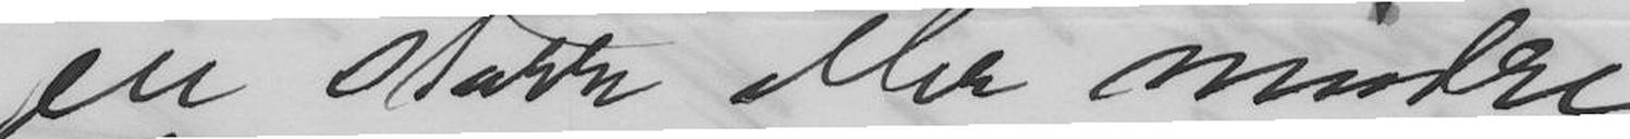en større eller mindre
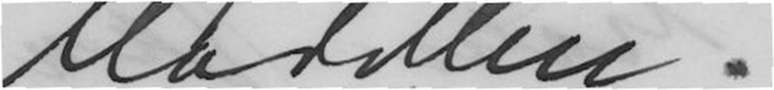Modellen. -
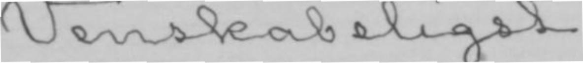Venskabeligst
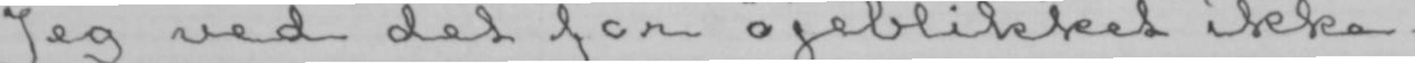Jeg ved det for øjeblikket ikke.
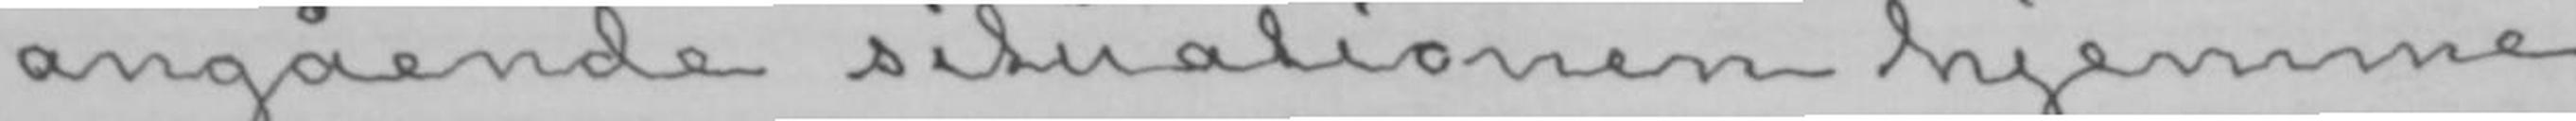angående situationen hjemme
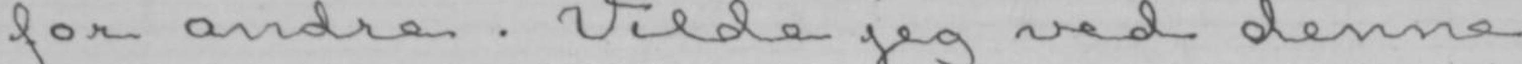for andre. Vilde jeg ved denne
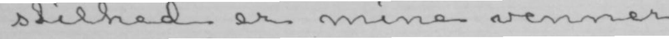stilhed er mine venner.
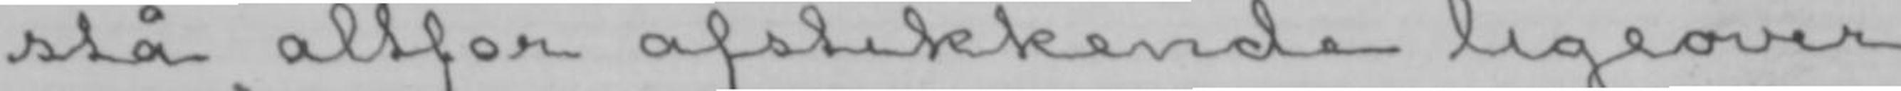stå altfor afstikkende ligeover
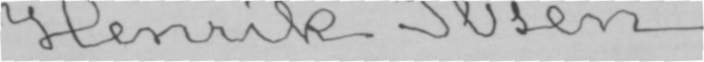Henrik Ibsen.
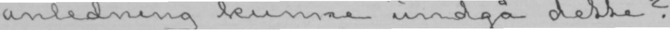anledning kunne undgå dette?
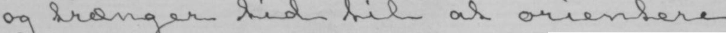og trænger tid til at orientere
